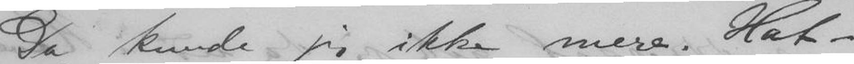Da kunde jeg ikke mere. Hat-
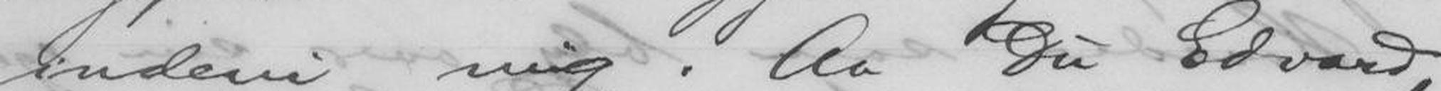indeni mig. Aa Du Edvard,
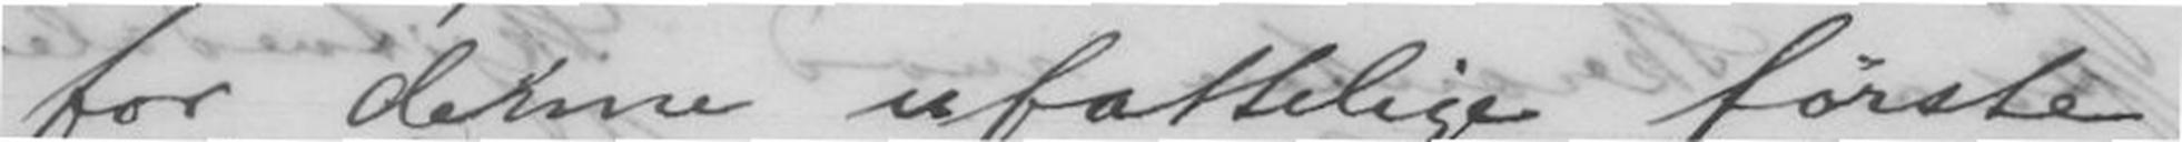for denne ufattelige første
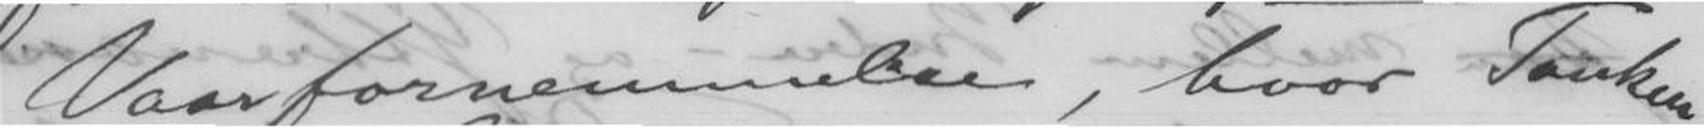Vaarfornemmelse, hvor Tanken
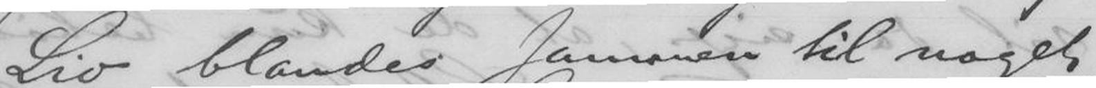Liv blandes sammen til noget,
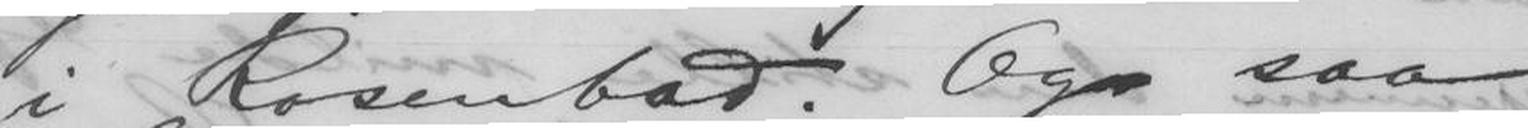i Rosenbad. Og saa
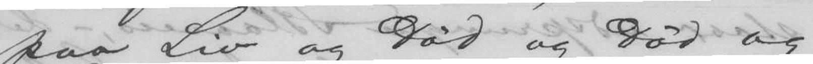paa Liv og Død og Død og
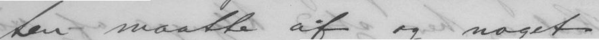ten maatte af og noget
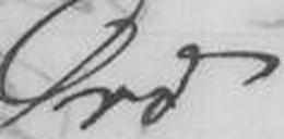Ord!
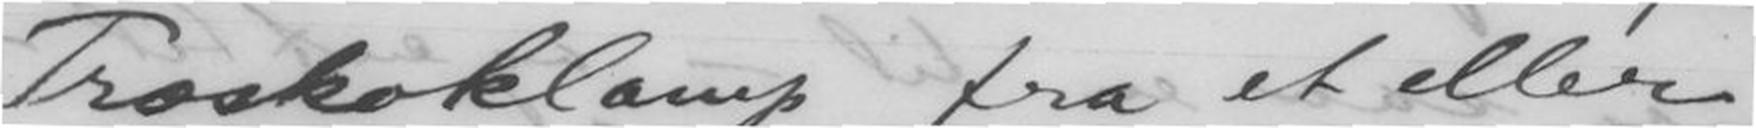Træskoklamp fra et eller
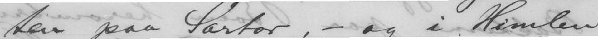ten paa Sartor, - og i Himlen
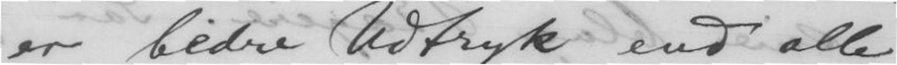er bedre Udtryk end alle
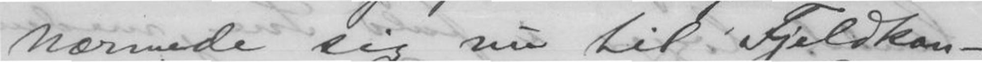nærmede sig nu til Fjeldkan-
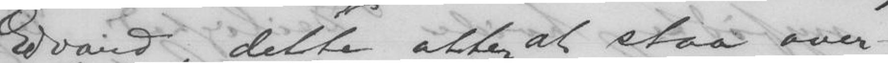Edvard, dette atter at staa over-
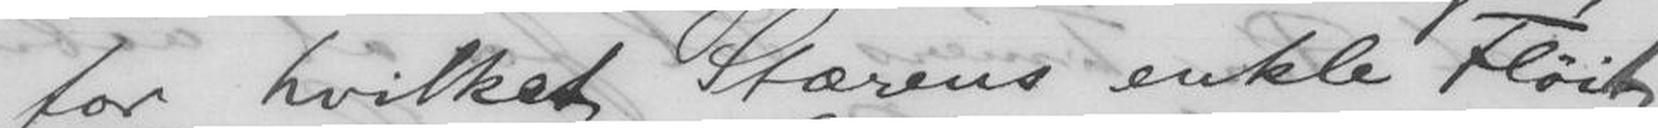for hvilket Stærens enkle Fløit
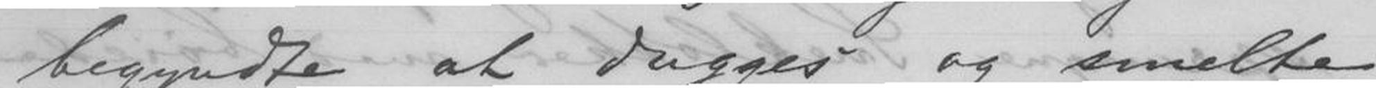begyndte at dugges og smelte
